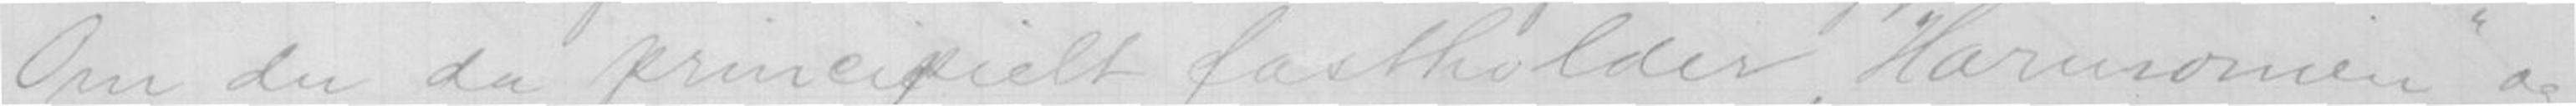Om du da principielt fastholder Harmonien og
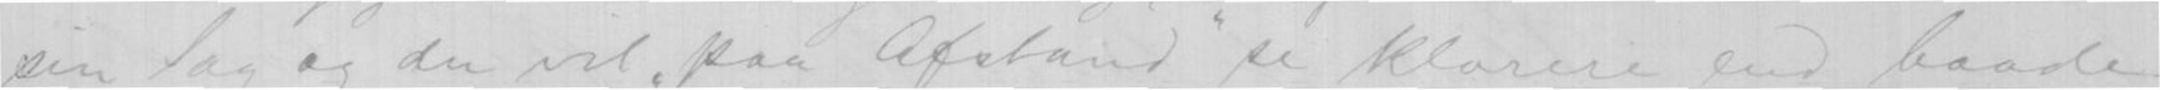sin Sag og du vil paa Afstand se klarere end baade
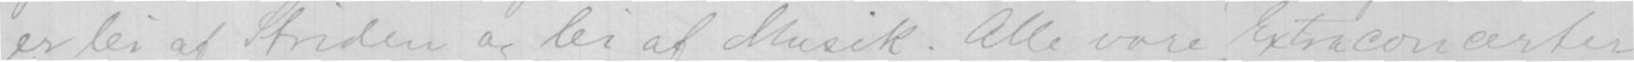er lei af Striden og lei af Musik. Alle vore Extraconcerter
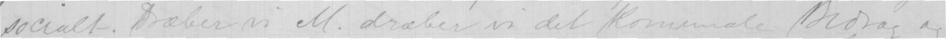socialt. Dræber vi M. dræber vi det komunale Bidrag og
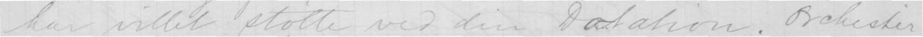har villet støtte ved din Dotation. Orchester.
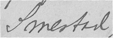Smestad
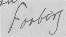Forberg
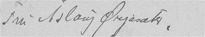Fru Åslaug Ørjasæter
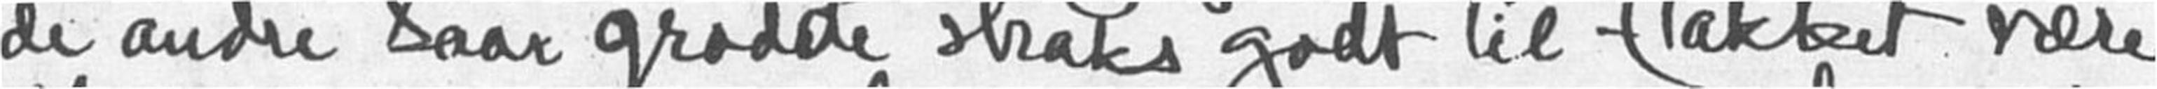de andre saar grodde straks godt til - (takket være
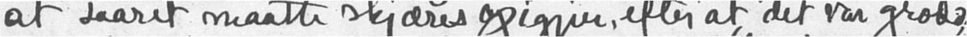at saaret maate skjæres op igjen, efter at det var grodd,
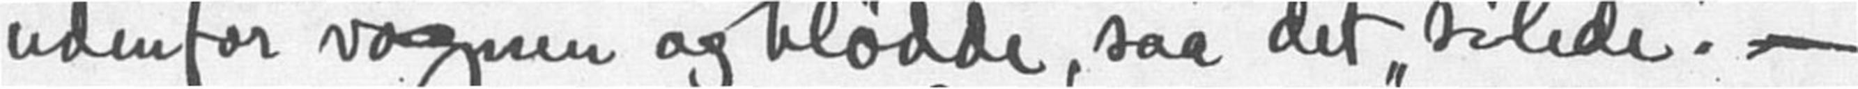udenfor vognene og blødde, saa det "silede". -
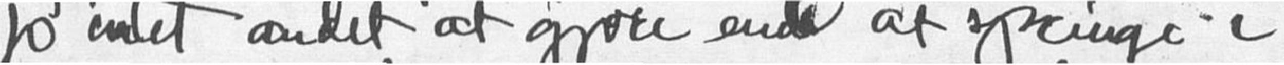jo intet andet at gjøre end at springe i
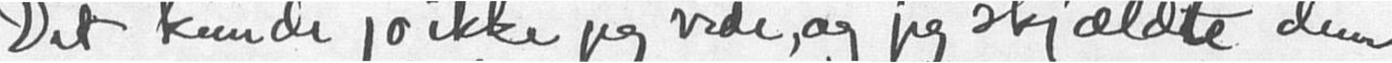Det kunde jo ikke jeg vide, og jeg skjældte dem
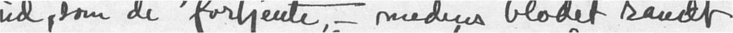ud, som de fortjente, - medens blodet randt
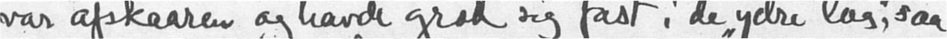var afskaaren og havde grod sig fast i de ydre "lag", saa
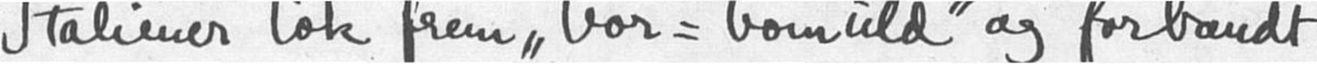Italiener tok frem "bor-bomuld" og forbandt
